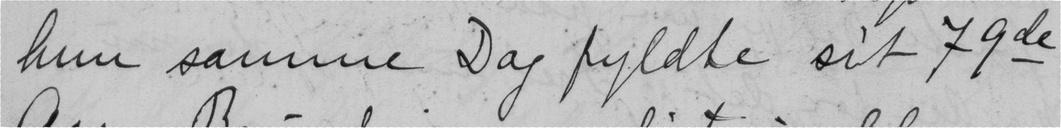hun samme Dag fyldte sit 79de
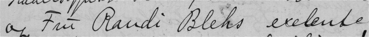og Fru Randi Blehs exelente
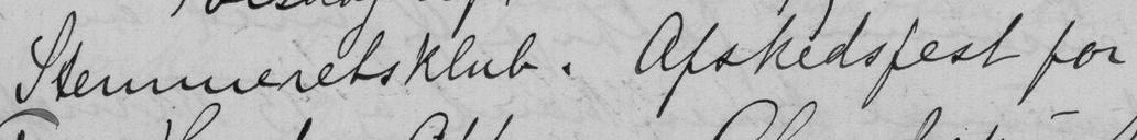Stemmeretsklub. Afskedsfest for
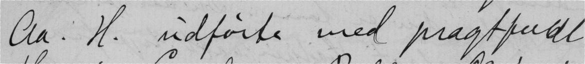Aa. H. udførte med pragtfuldt
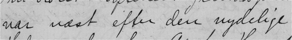var næst efter den nydelige
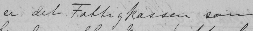er det Fattigkassen som
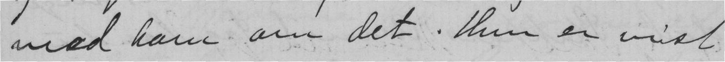med ham om det. Hun er vist
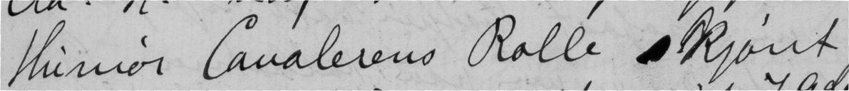Humør Cavalerens Rolle skjønt
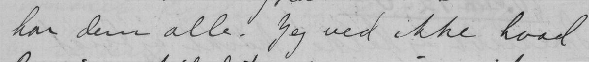har dem alle. Jeg ved ikke hvad
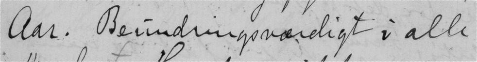aar. Beundringsværdigt i alle
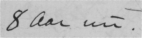8 aar nu.
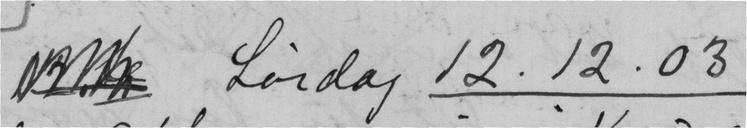Lørdag 12.12.03
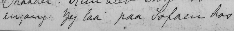engang. Jeg laa paa Sofaen hos
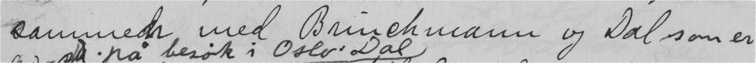sammen med Brinchmann og Dal som er
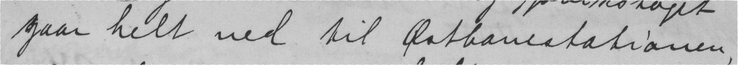gaar helt ned til Østbanestationen,
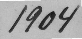1904
